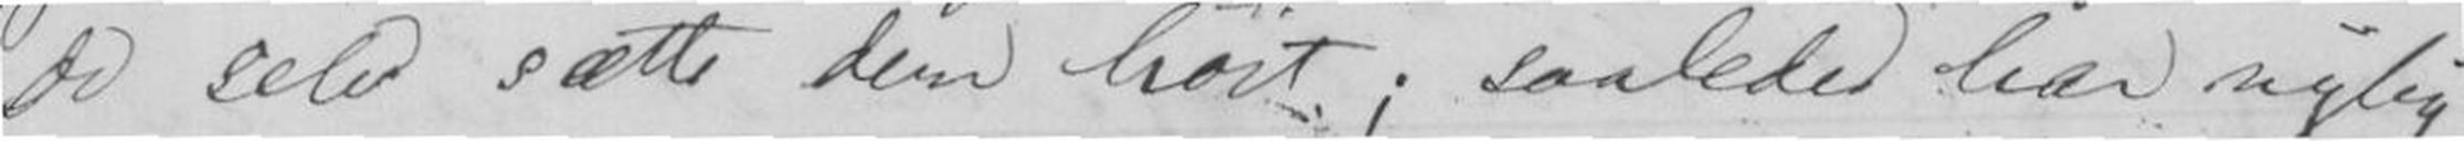Du selv satte dem høit; saaledes har nylig
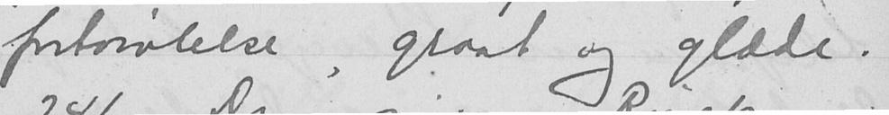fortvivlelse, graat og glæde.
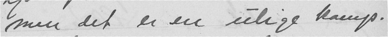men det er en ulige kamp.
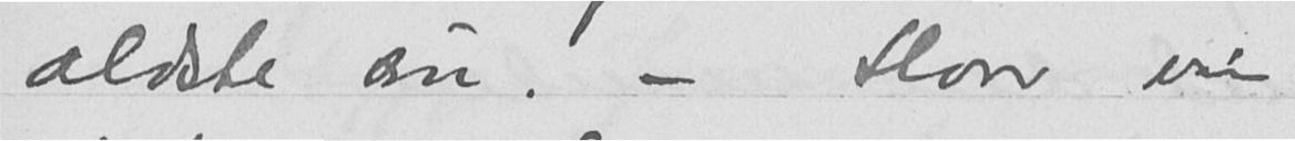ældste søn. - Hvor var
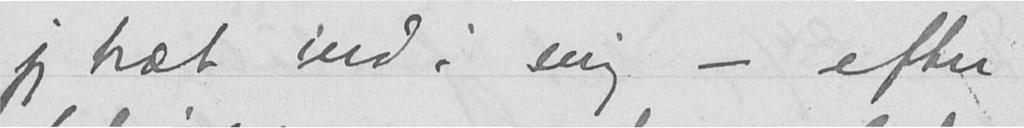jeg træt ind i mig - efter
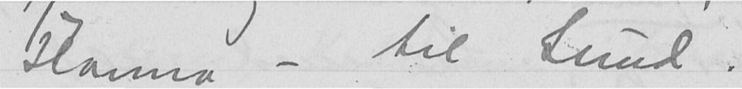Hanna - til Sund.
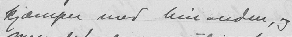kjæmper med hinanden, og
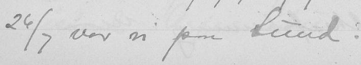26/7 var vi paa Sund.
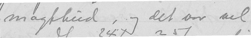magtbud, og det var vel
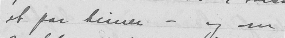et par timer - og om
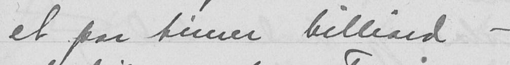et par timer billiard -
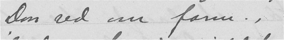Don red om form.,
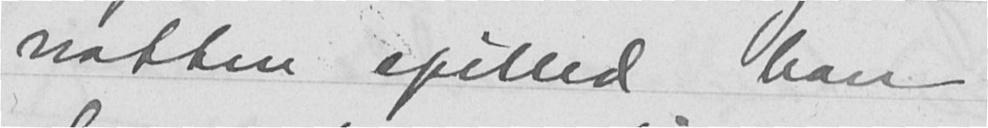natten spilled han
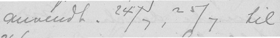anvendt. 24/7, 25/7 til
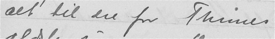alt til ære for Thrines
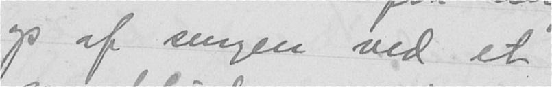op af sengen ved et
